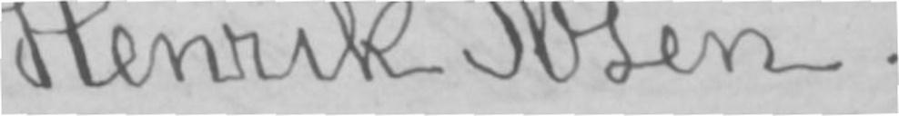Henrik Ibsen.
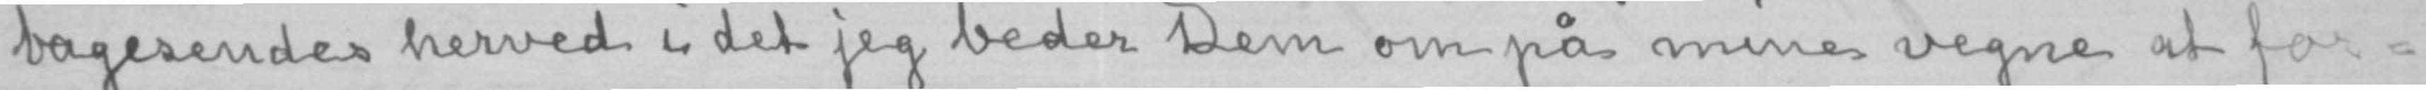bagesendes herved i det jeg beder Dem om på mine vegne at for-
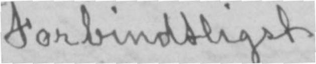Forbindtligst
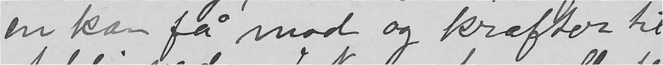en kan få mod og kræfter til
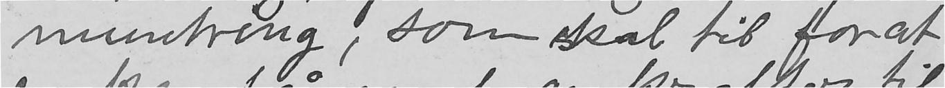muntring, som skal til forat
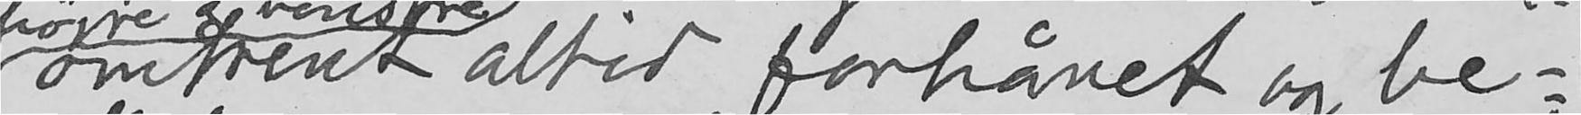omtrent altid forhånet og be-
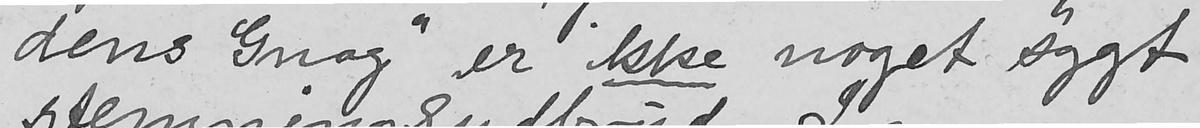dens Gnag" er ikke noget sygt
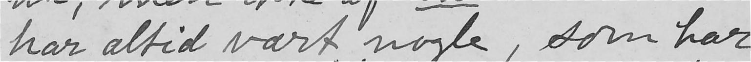har altid vært nogle, som har
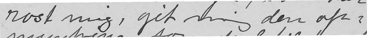rost mig, git mig den op-
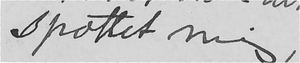spottet mig,
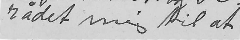rådet mig til at
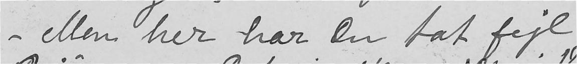- Men her har Du tat fejl
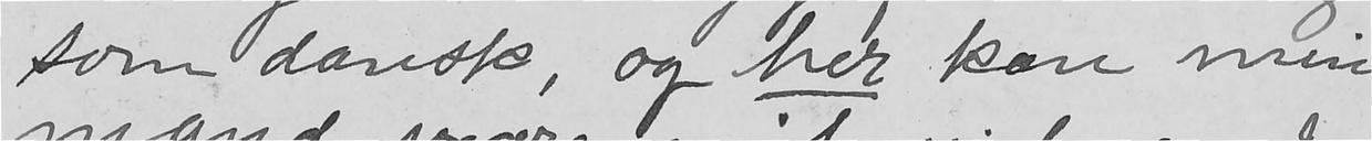som dansk, og her kan min
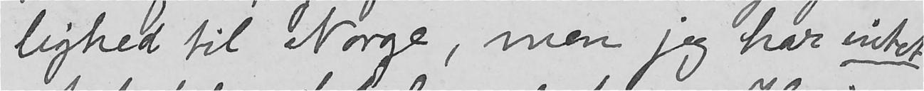lighed til Norge, men jeg har intet
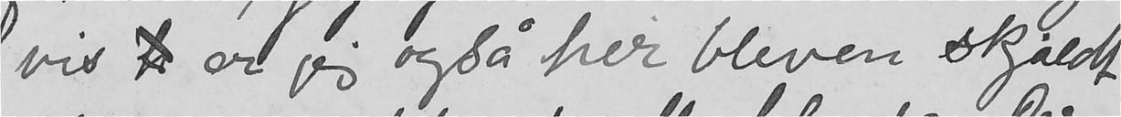vis er jeg også her bleven skjældt
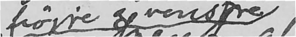højre og venstre
